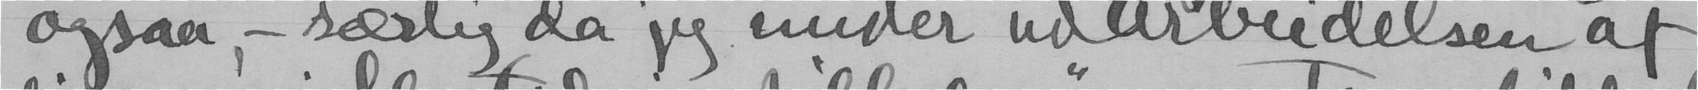ogsaa, - særlig da jeg under udarbeidelsen af
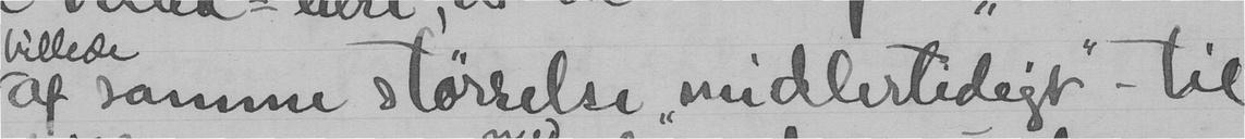af samme størrelse "midlertidigt" - til
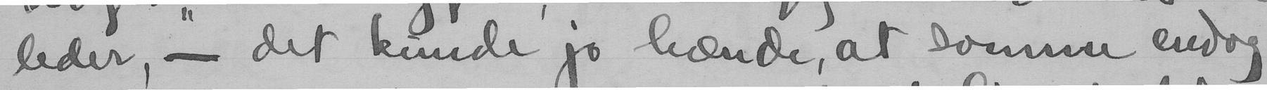leder, - det kunde jo hænde, at somme endog
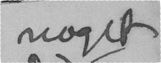noget
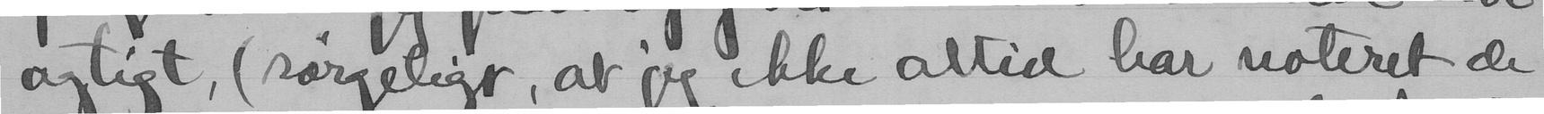agtigt, (sørgeligt, at jeg ikke altid har noteret de
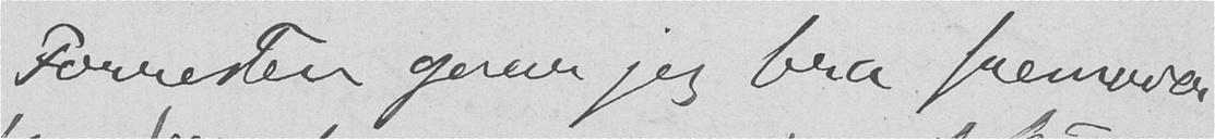Forresten gaar jeg bra fremover
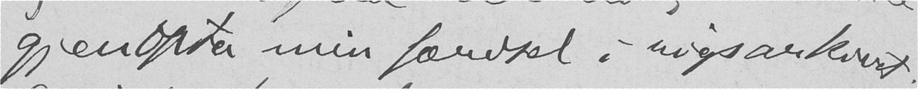gjenopta min færdsel i rigsarkivet.
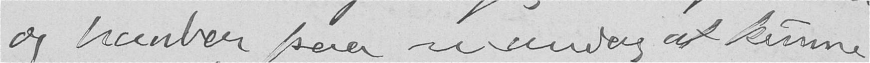og haaber paa mandag at kunne
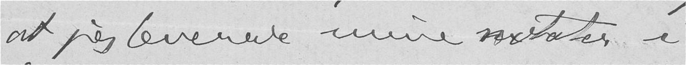at jeg leverede mine notater i
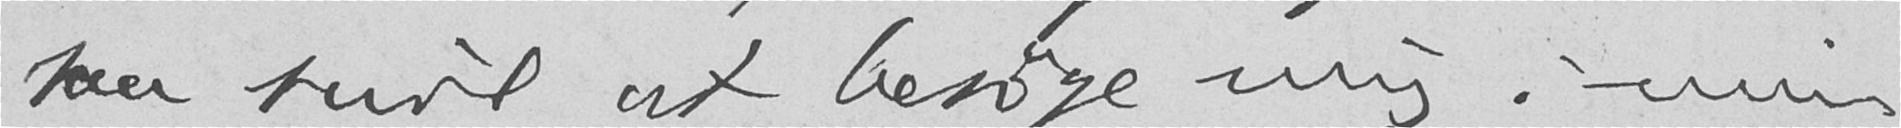saa snil at besøge mig i min
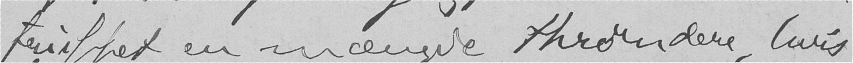truffet en mængde thrøndere, hvis
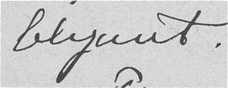blyant.
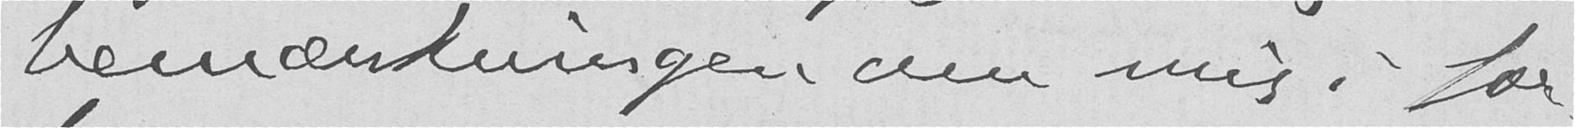bemærkningen om mig i for-
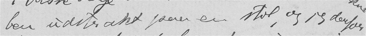ben udstrakt paa en stol, og jeg derfor
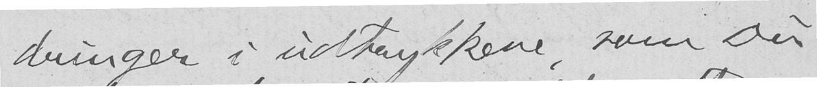dringer i udtrykkene, som Du
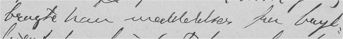bragte han meddelelser fra bryl-
